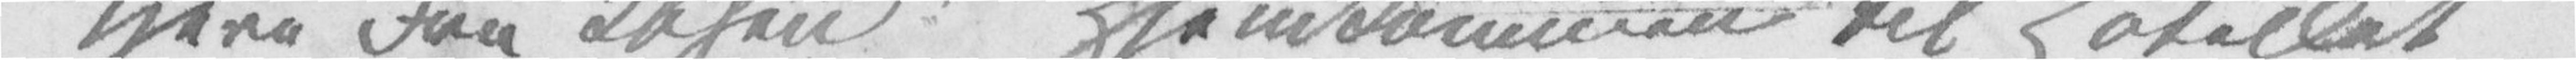Kjere Fru Ibsen! Hjemkommen til Hotellet
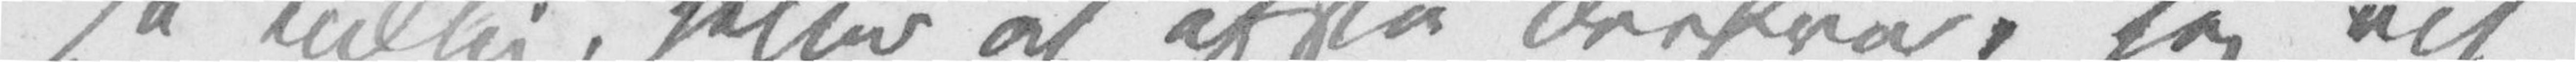så tidlig, heller at afstå derfra, jeg vil
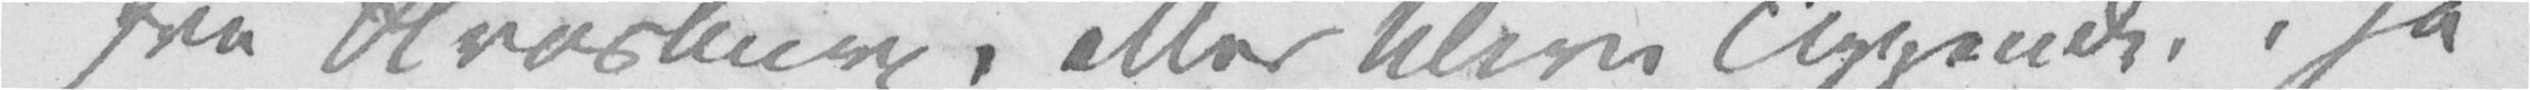fra Strasburg, eller blive liggende, i så
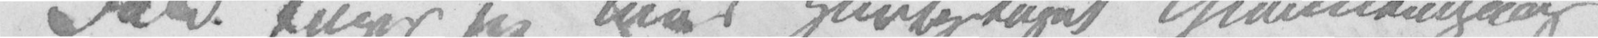Fald tager jeg med Hurtigtoget Giennemgangs
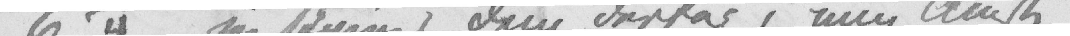6 ¼, jeg skriver Dem derfor i min Angst
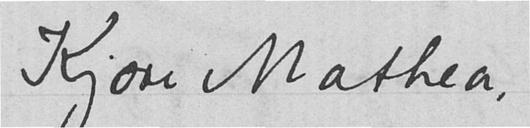Kjære Mathea,
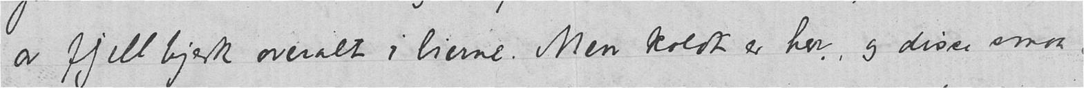av fjellbjerk overalt i lierne. Men koldt er her, og disse smaa
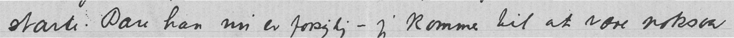starte. Bare han nu er forsigtig – jeg kommer til at være noksaa
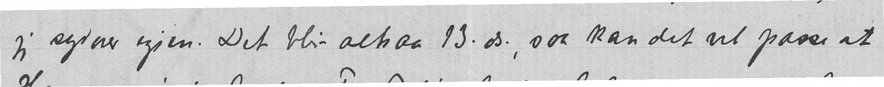jeg sydover igjen. Det blir altsaa 13. ds., saa kan det vel passe at
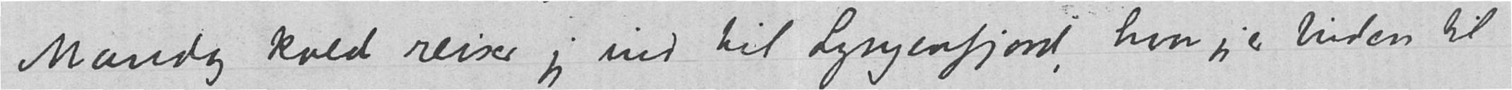Mandag kveld reiser jeg ind til Lyngenfjord, hvor jeg er buden til
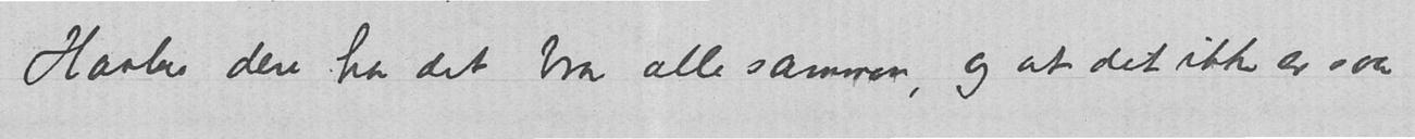Haaber dere har det bra alle sammen, og at det ikke er saa
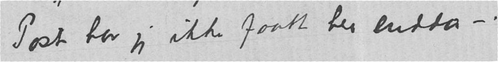Post har jeg ikke faatt her endda –.
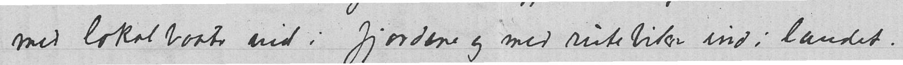med lokalbaater ind i fjordene og med rutebiler ind i landet.
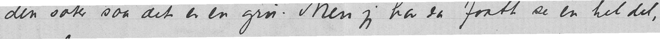den soter saa det er en gru. Men jeg har da faatt se en hel del,
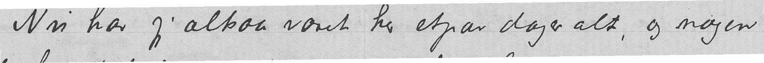Nu har jeg altsaa været her etpar dager alt, og nogen
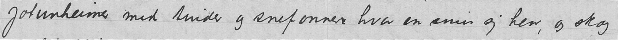Jotunheimen med tinder og snefonner hvor en snur sig hen, og skog
